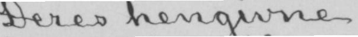Deres hengivne
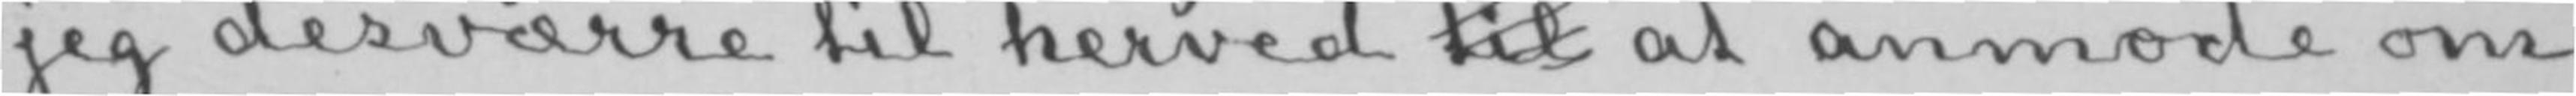jeg desværre til herved til at anmode om
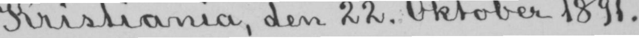Kristiania, den 22. Oktober 1891.
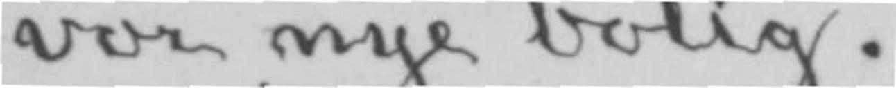vor nye bolig.
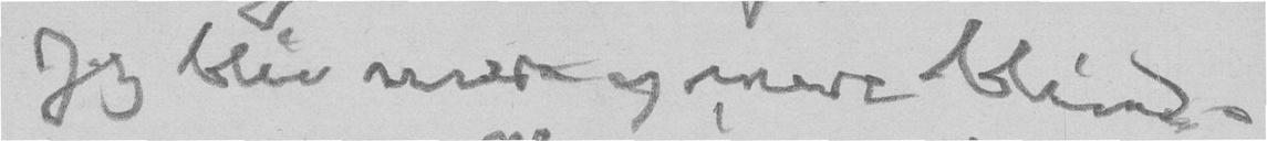Jeg blir mere og mere blind.
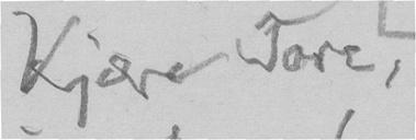Kjære Tore,
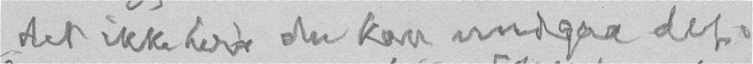det ikke hvis du kan undgaa det.
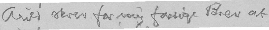Arild skrev for mig forrige Brev at
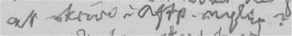at skrive i Aftp. nylig?
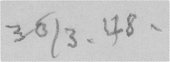30/3. 48.
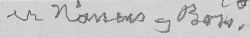er Nonsens og Boss.
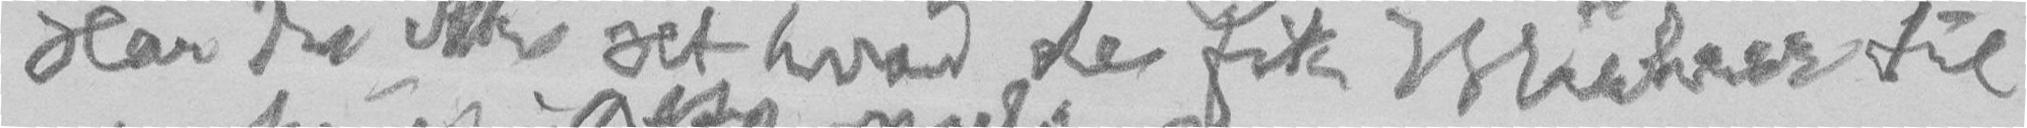Har du ikke set hvad de fik Mührer til
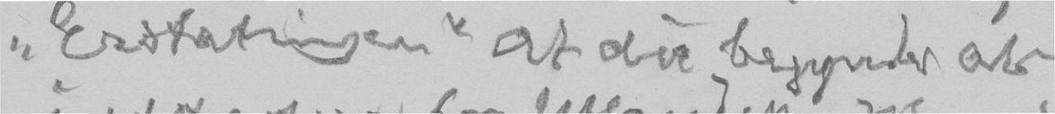"Erstatningen" at du begynder at
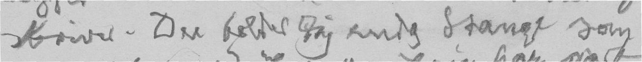skriver. Du holder dig endog Stange som
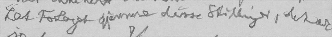Lat Forlaget gjemme disse Skillinger, det er
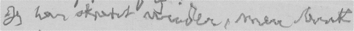Jeg har skrevet under, men bruk
